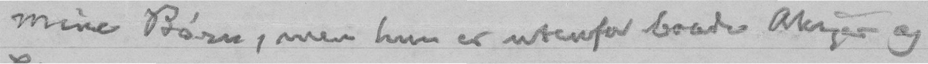mine Børn, men hun er utenfor baade Aksjer og
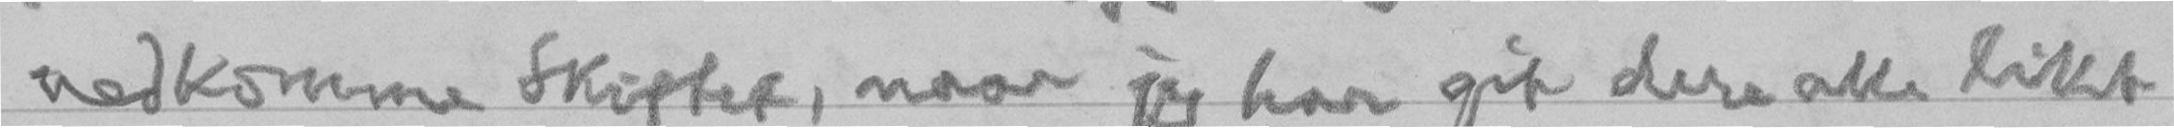vedkomme Skiftet, naar jeg har git dere alle likt
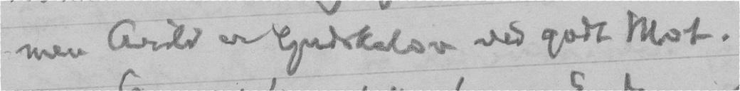men Arild er Gudskelov ved godt Mot.
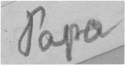Papa
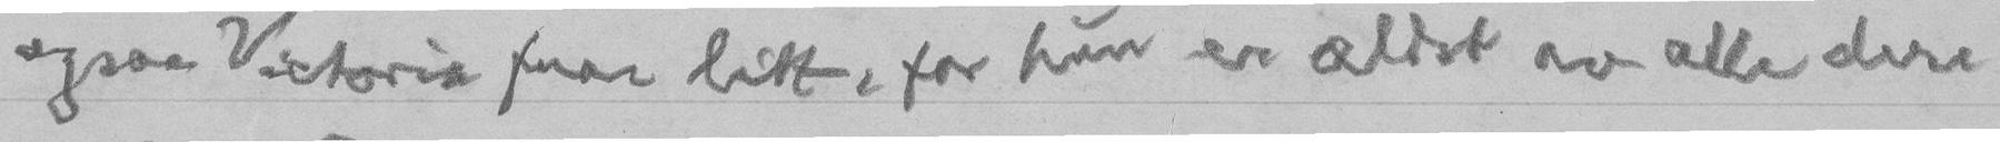ogsaa Victoria faar litt, for hun er ældst av alle dere
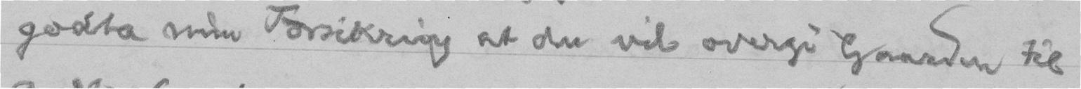godta min Forsikring at du vil overgi Gaarden til
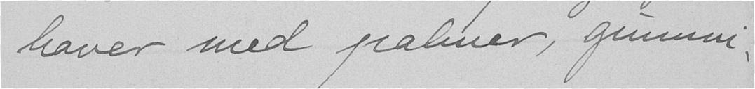haver med palmer, gummi
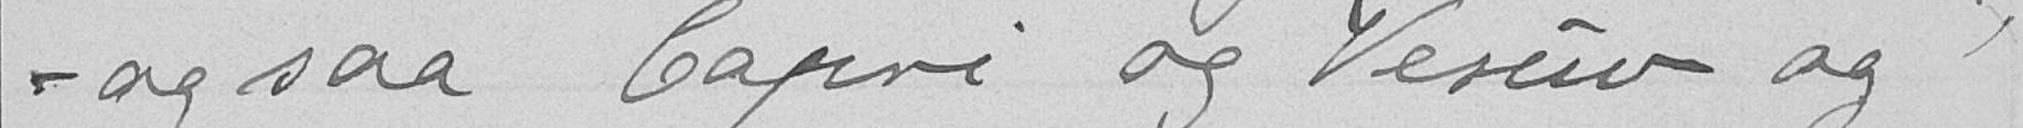- og saa Capri og Vesuv og
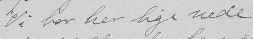Vi bor her lige nede
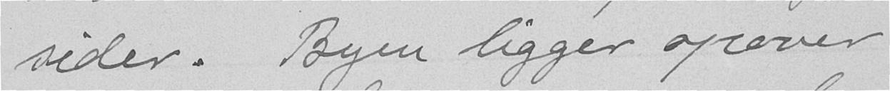sider. Byen ligger opover
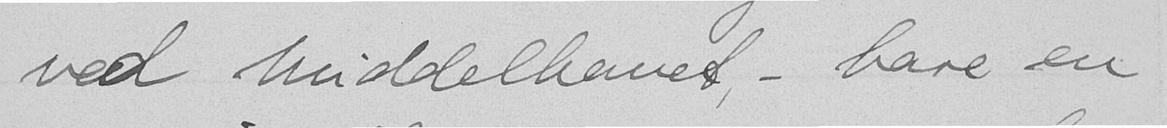ved Middelhavet,- bare en
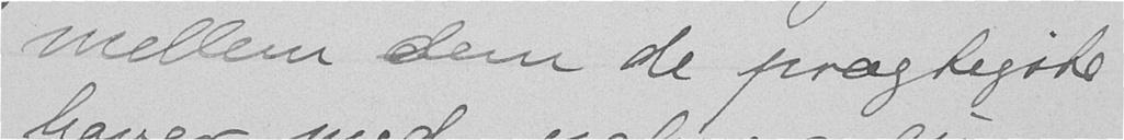mellem dem de prægtigste
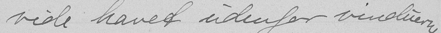vide havet udenfor vinduerne
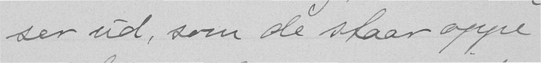ser ud, som de staar oppe
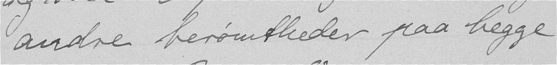andre berømtheder paa begge
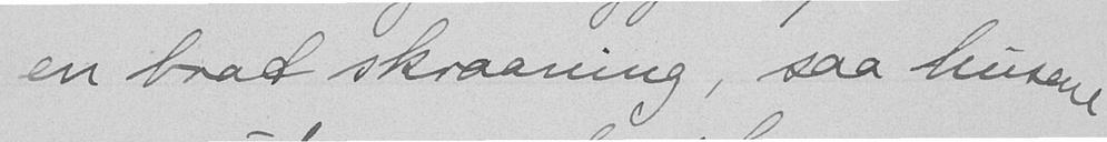en brat skraaning, saa husene
